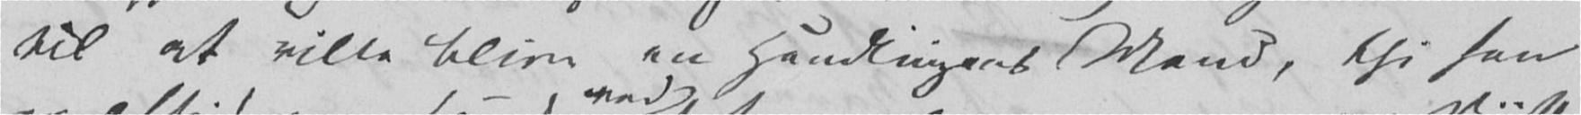til at ville blive en Handlingens Mand, thi han
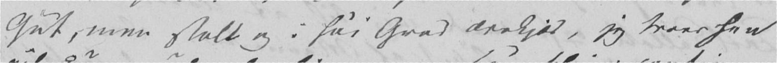Gut, men stolt og i høi Grad ærekjær, jeg troer han
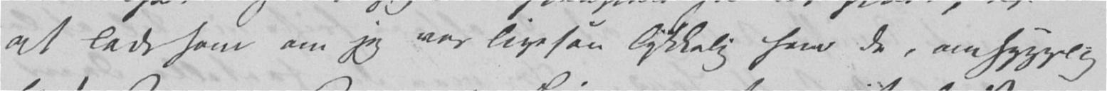at lade som om jeg var ligesaa lykkelig som de, omhyggelig
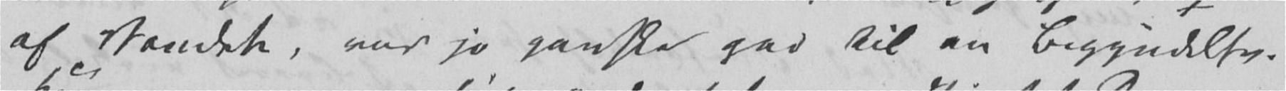af Vandet, var jo ganske god til en Begyndelse.
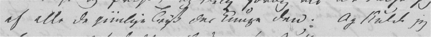af alle de piinlige Tryk der knuge den. Og skulde jeg
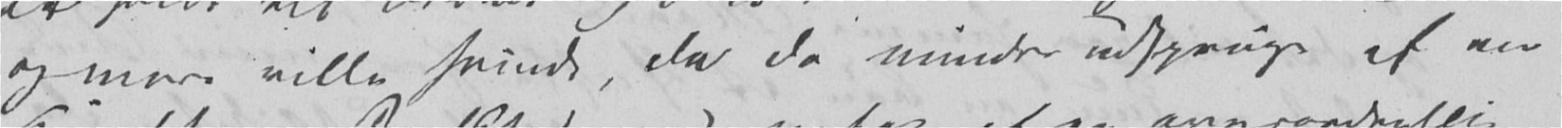og mere ville svinde, da de mindre udspringe af en
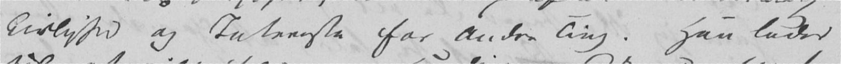Livlighed og Interesse for Andre Ting. Han lader
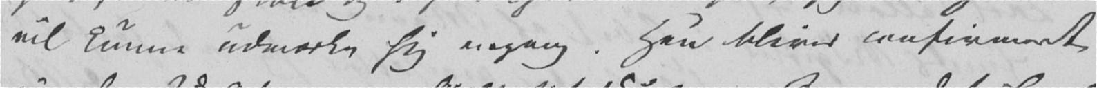vil kunne udmærke sig engang. Han bliver confirmeret
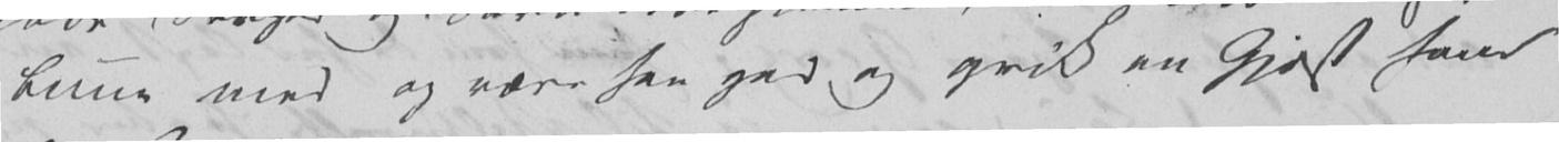Lune med og være saa god og qvik en Gjæst som
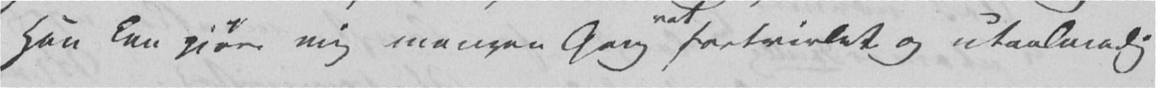Han kan gjøre mig mangen Gang fortvivlet og utaalmodig
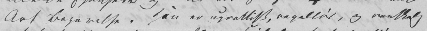Art Begavelse. Han er upraktisk, regelløs, og vanskelig
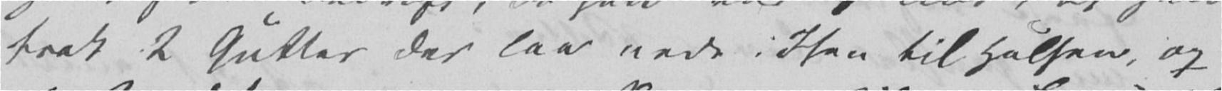trak 2 Gutter der laa nede i Isen til Halsen, op
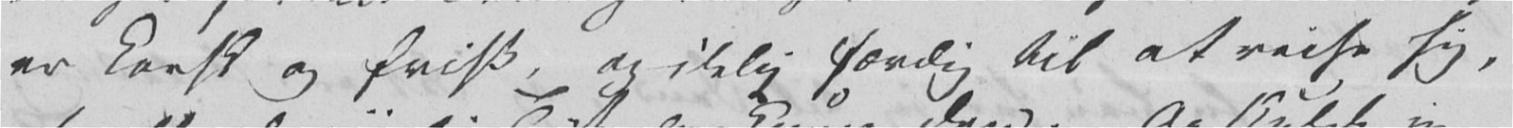er karsk og frisk, og idelig færdig til at reise sig,
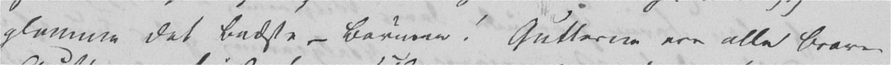glemme det Bedste – Børnene! Gutterne ere alle brave
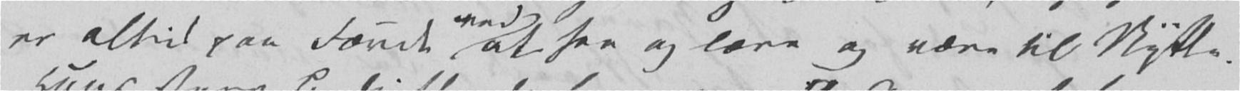er altid paa Færde at see og lære og være til Nytte.
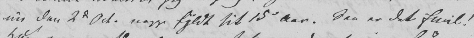nu den 2den Oct. neppe fyldt sit 15de Aar. Saa er det Emil!
